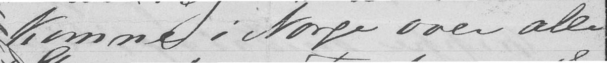komne i Norge over alle
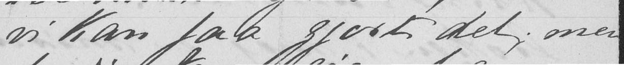vi kan faa gjort det; men
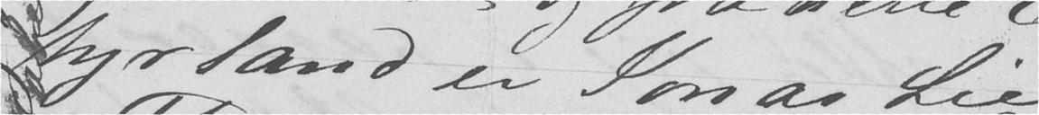tyrland er Jonas Lie
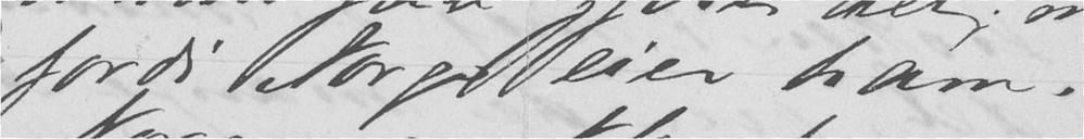fordi Norge eier ham.
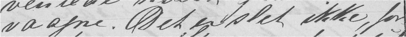vaagne. Det er slet ikke, for-
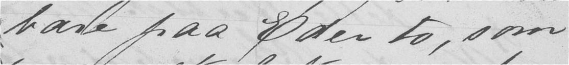bare paa Eder to, som
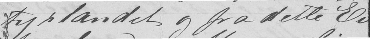tyrlandet og fra dette Ev
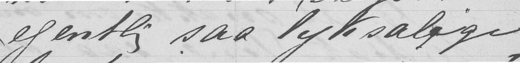egentlig saa lyksalige
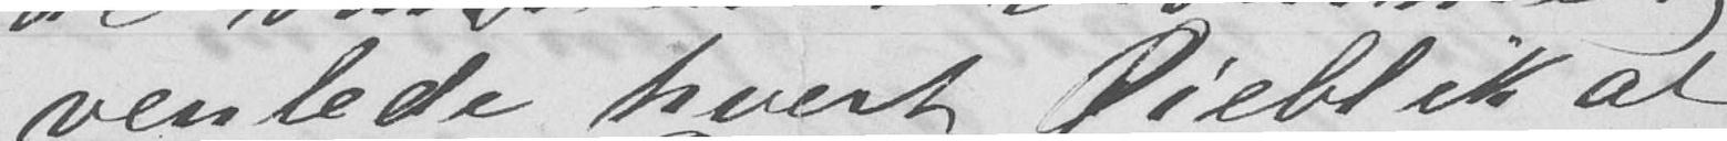ventede hvert Øieblik at
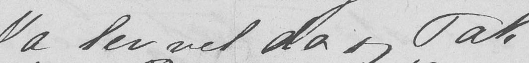Ja lev vel da og Tak
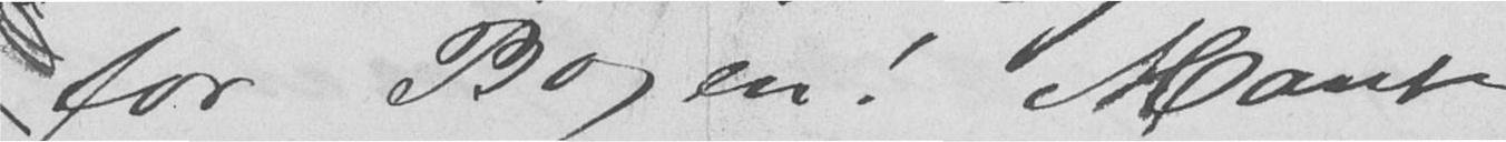for Bogen! Mange
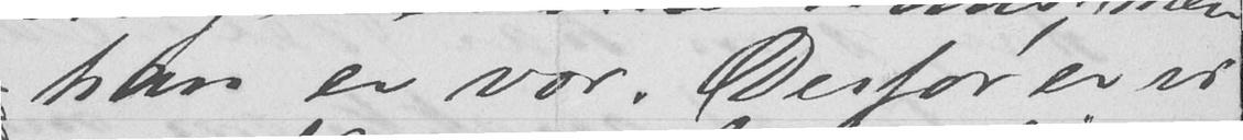han er vor. Derfor er vi
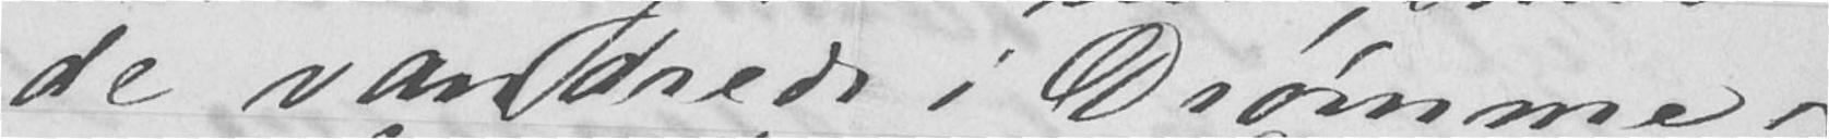de vandrede i Drømme og
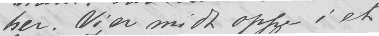her. Vi er midt oppe i et
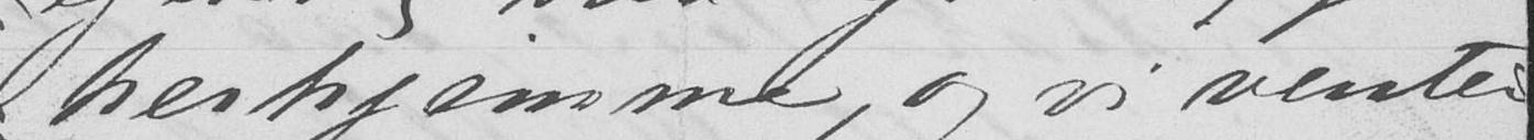herhjemme, og vi venter
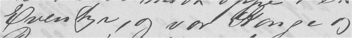Eventyr, og vor Konge og
